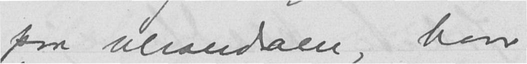paa verandaen, hvor
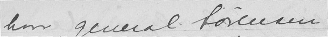hvor general Sørensen
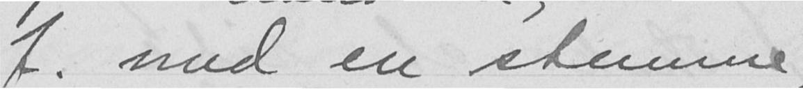J. med en stemme,
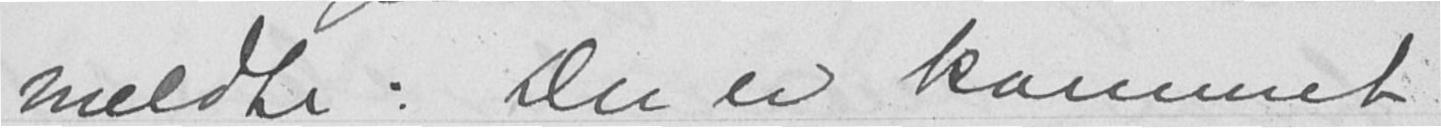meldte: Der er kommet
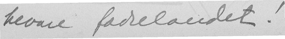bevare fædrelandet!
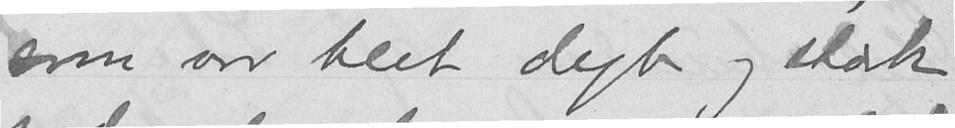som var blit dyb og stærk
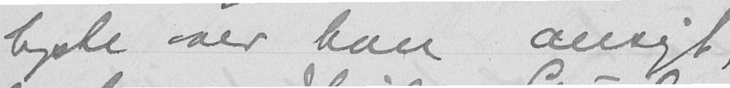lyste over hans ansigt,
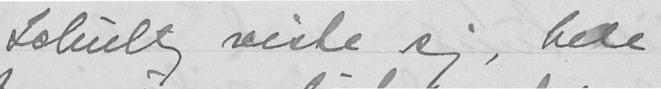Schultz reiste sig, hele
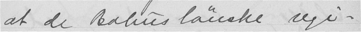at de Bohuslänske regi-
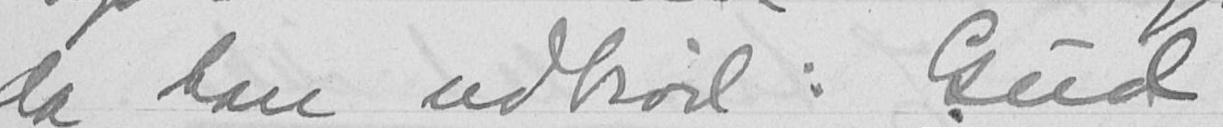da han udbrød: Gud
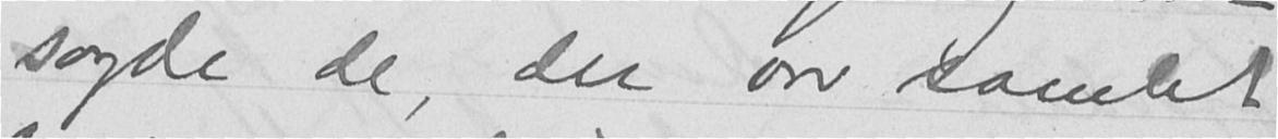sagde de, der var samlet,
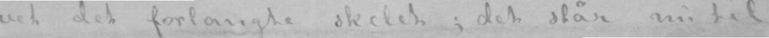vet det forlangte skelet; det står nu til
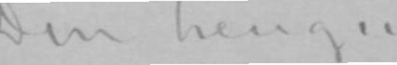Din hengivne
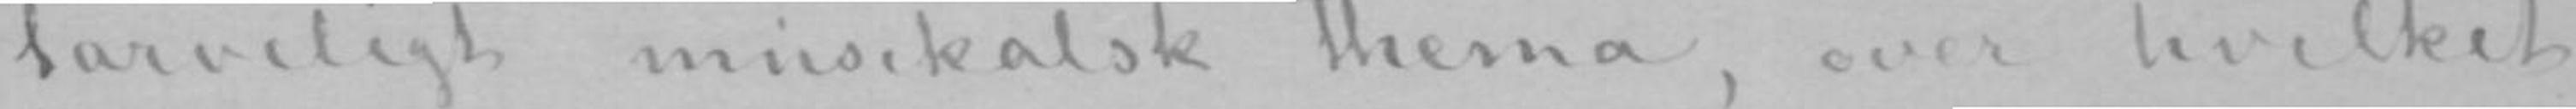tarveligt musikalsk thema, over hvilket
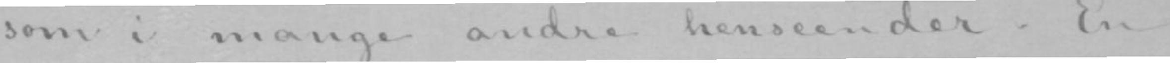som i mange andre henseender. En
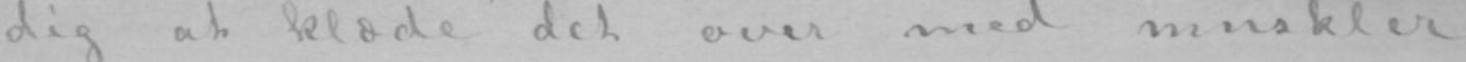dig at klæde det over med muskler
